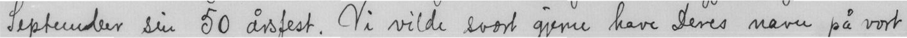September sin 50 årsfest. Vi vilde svært gjerne have Deres navn på vort
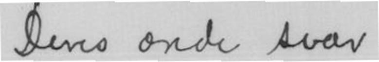Deres ærede svar
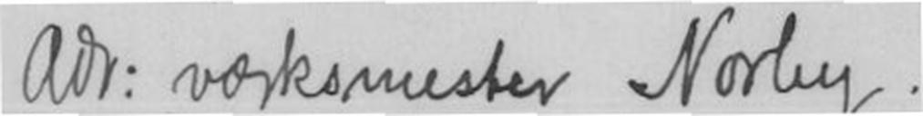Adr: værksmester Norby,
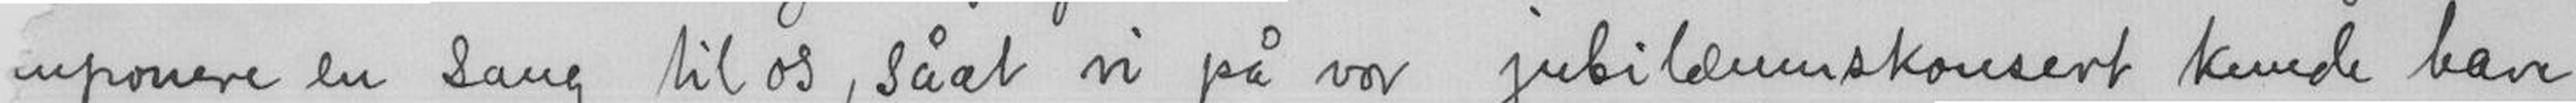mponere en sang til os, såat vi på vor jubilæumskonsert kunde have
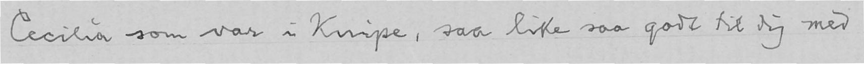Cecilia som var i Knipe, saa like saa godt til dig med
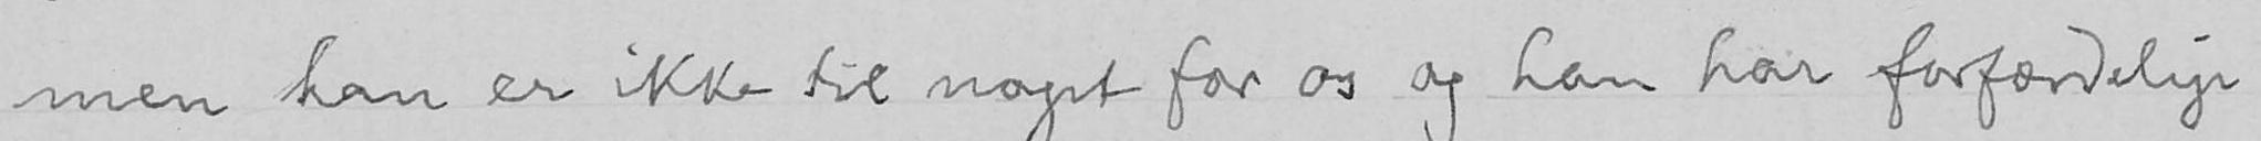men han er ikke til noget for os og han har forfærdelige
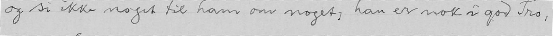og si ikke noget til ham om noget, han er nok i god Tro,
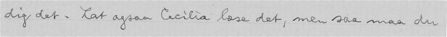dig det. Lat ogsaa Cecilia læse det, men saa maa du
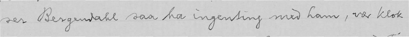ser Bergendahl saa ha ingenting med ham, vær klok
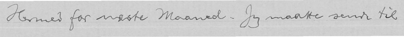Hermed for næste Maaned. Jeg maatte sende til
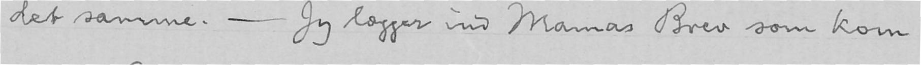det samme. - Jeg lægger ind Mamas Brev som kom
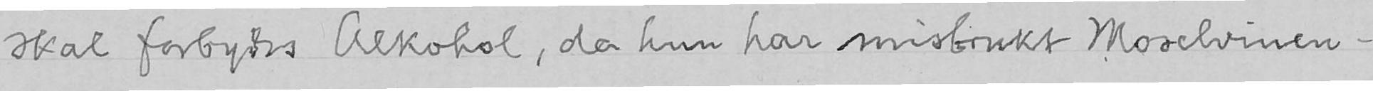skal forbydes Alkohol, da hun har misbrukt Moselvinen.
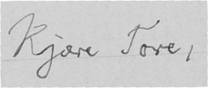Kjære Tore,
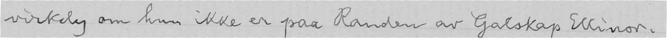virkelig om hun ikke er paa Randen av Galskap Ellinor.
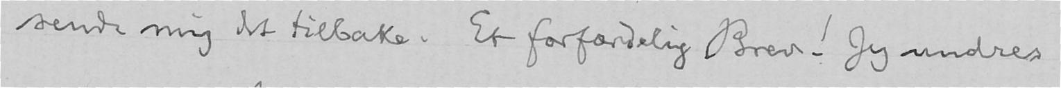sende mig det tilbake. Et forfærdelig Brev! Jeg undres
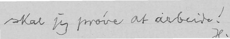skal jeg prøve at arbeide!
